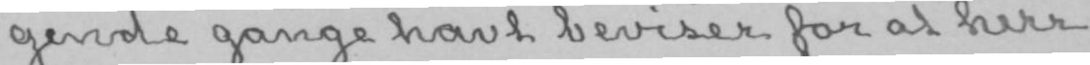gende gange havt beviser for at herr
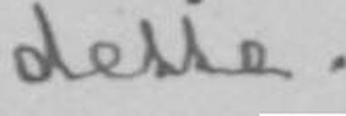dette.
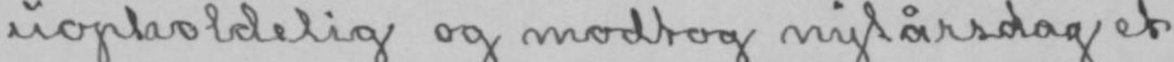uopholdelig og modtog nytårsdag et
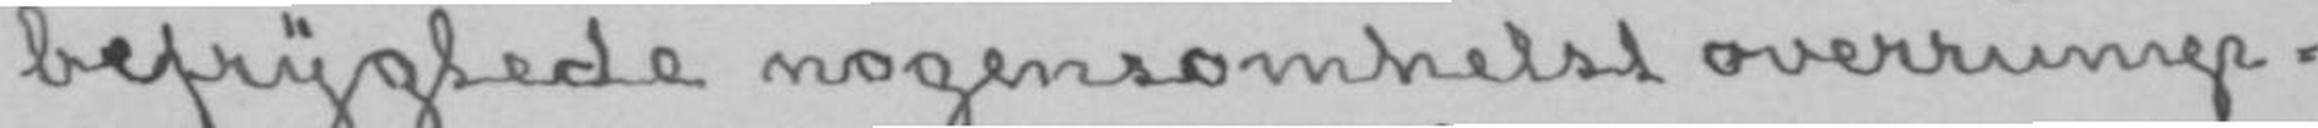befrygtede nogensomhelst overrump-
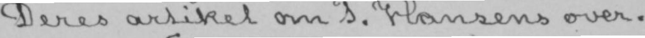Deres artikel om P. Hansens over-
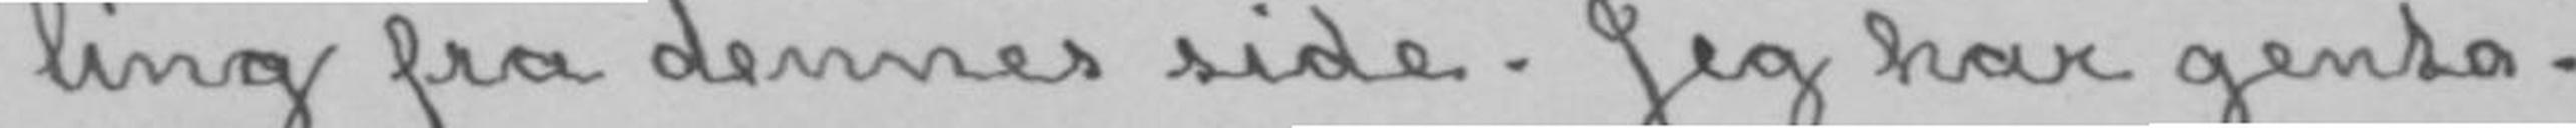ling fra dennes side. Jeg har genta-
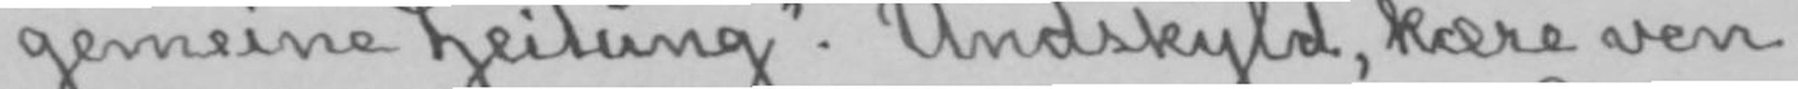gemeine Zeitung». Undskyld, kære ven,
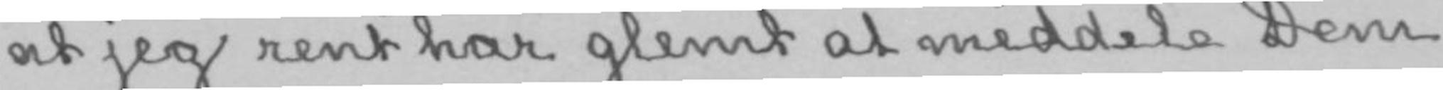at jeg rent har glemt at meddele Dem
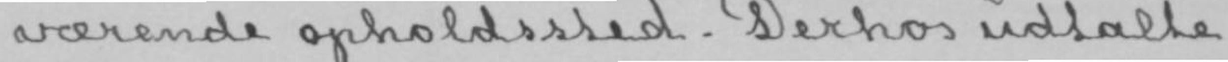værende opholdssted. Derhos udtalte
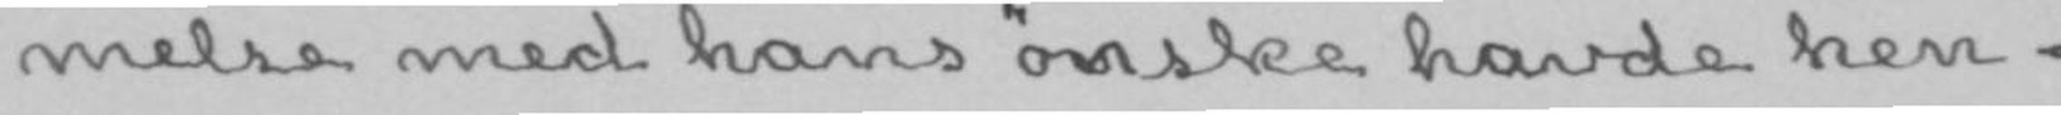melse med hans ønske havde hen-
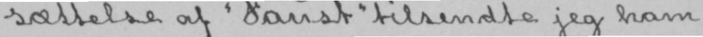sættelse af «Faust» tilsendte jeg ham
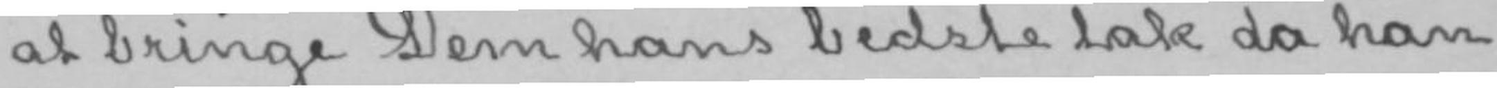at bringe Dem hans bedste tak da han
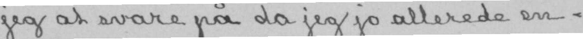jeg at svare på da jeg jo allerede en-
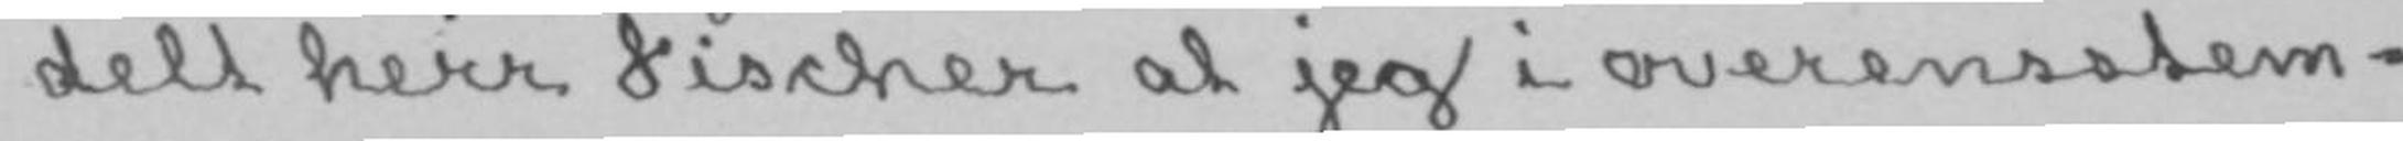delt herr Fischer at jeg i overensstem-
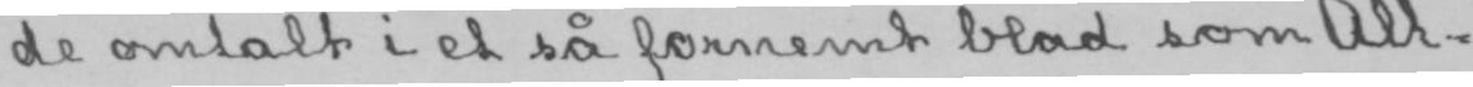de omtalt i et så fornemt blad som All-
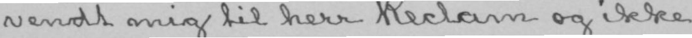vendt mig til herr Reclam og ikke
